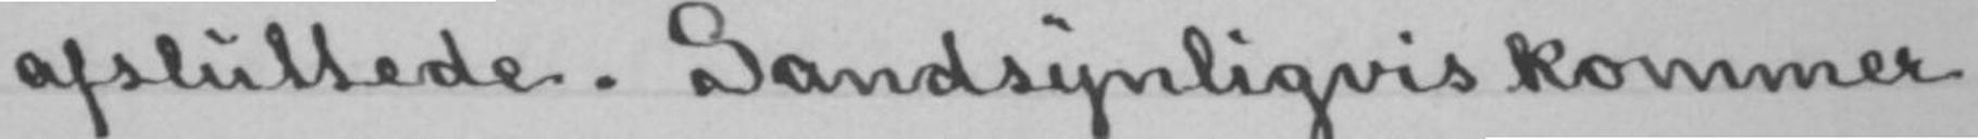afsluttede. Sandsynligvis kommer
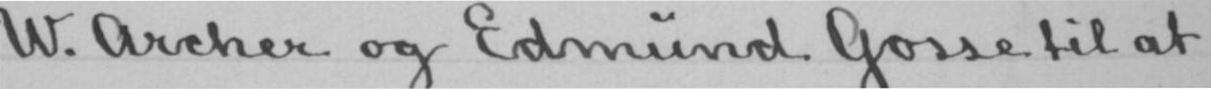W. Archer og Edmund Gosse til at
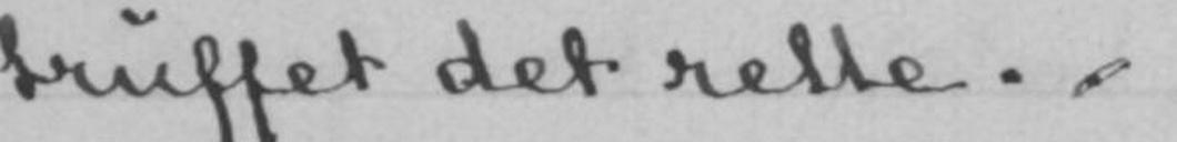truffet det rette.
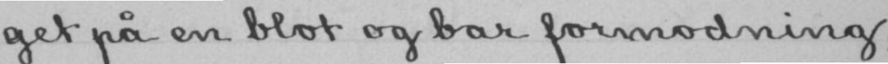get på en blot og bar formodning,
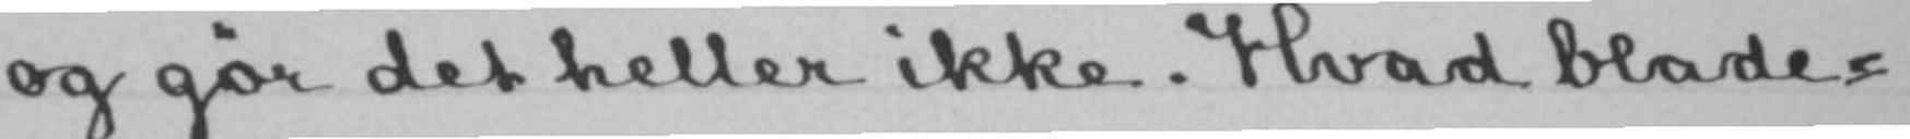og gør det heller ikke. Hvad blade-
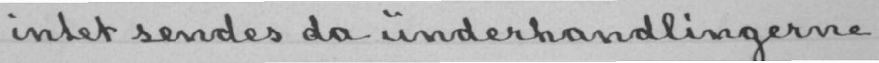intet sendes da underhandlingerne
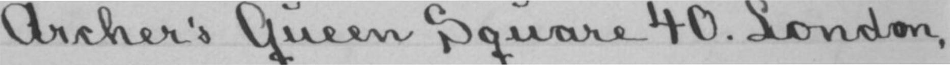Archer's Queen Square 40. London,
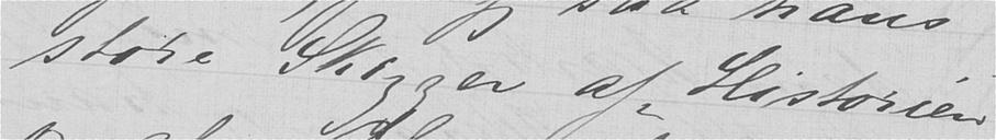store Skizzer af Historien
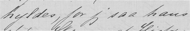hyldes, for jeg saa hans
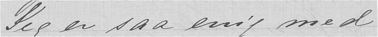Jeg er saa enig med
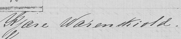Kjære Wærenskiold.
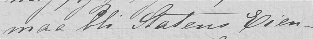maa bli Statens Eien-
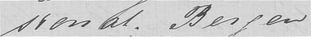sionat. Bergen
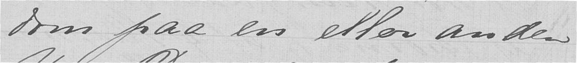dom paa en ellar anden
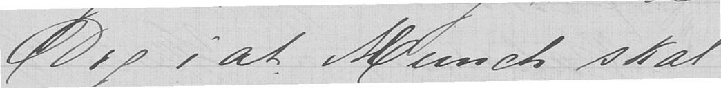Dig i at Munch skal
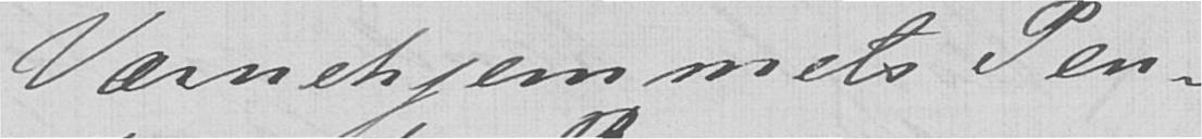Værnehjemmets Pen-
